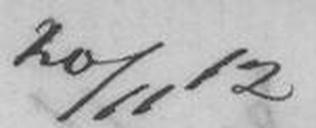20/11 12.
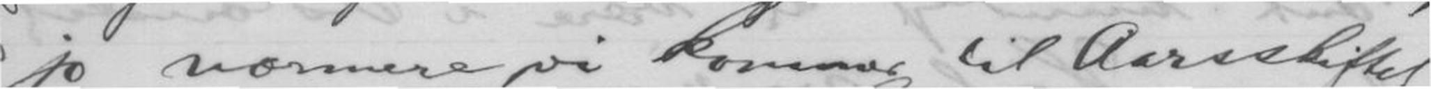jo nærmere vi kommer til Aarsskiftet,
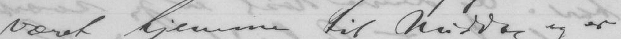været hjemme til Middag og er
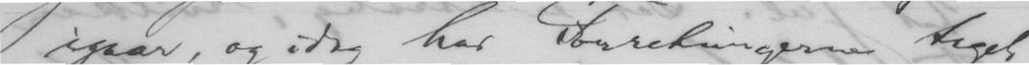igaar, og idag har Forretningerne taget
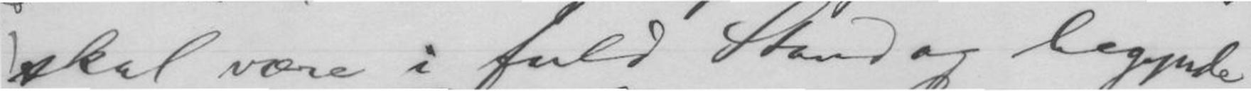skal være i fuld Stand og begynde
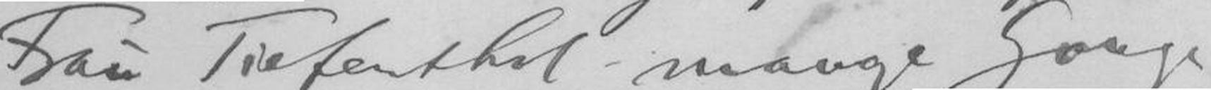Frau Tiefenthal mange gange
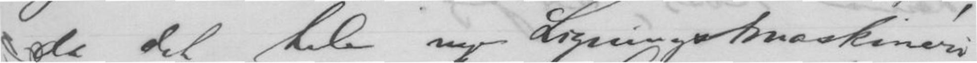da det hele nye Ligningsmaskineri
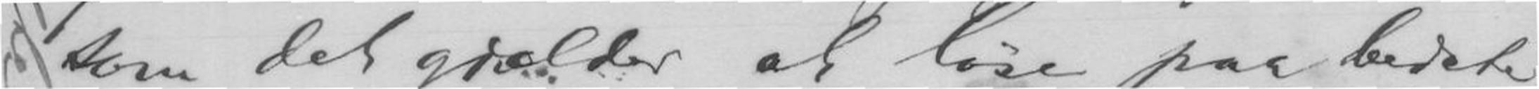som det gjælder at løse paa bedste
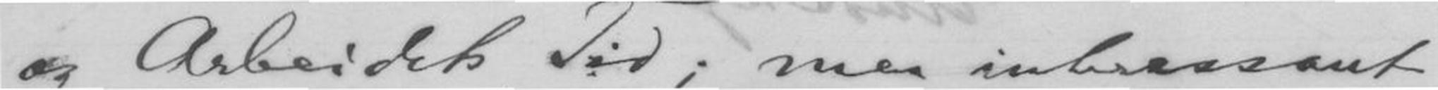og Arbeidets Tid; men interæssant,
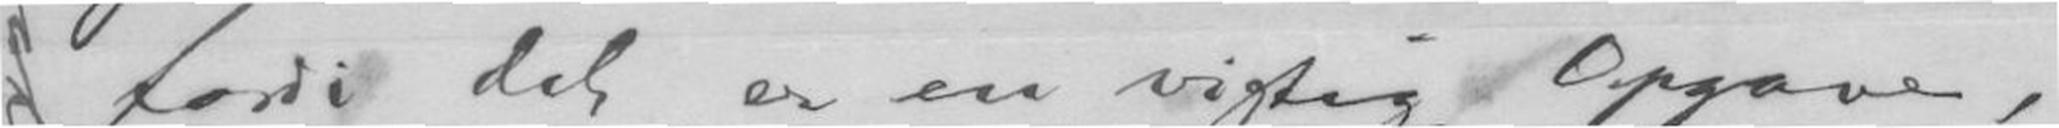fordi det er en vigtig Opgave,
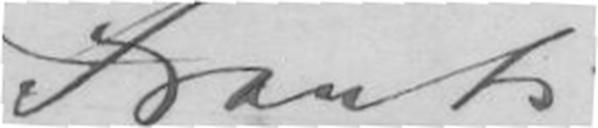Frants
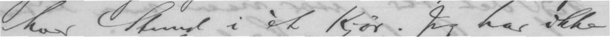hver Stund i et Kjør. Jeg har ikke
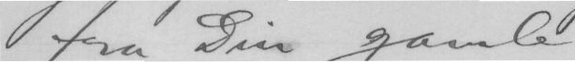fra Din gamle
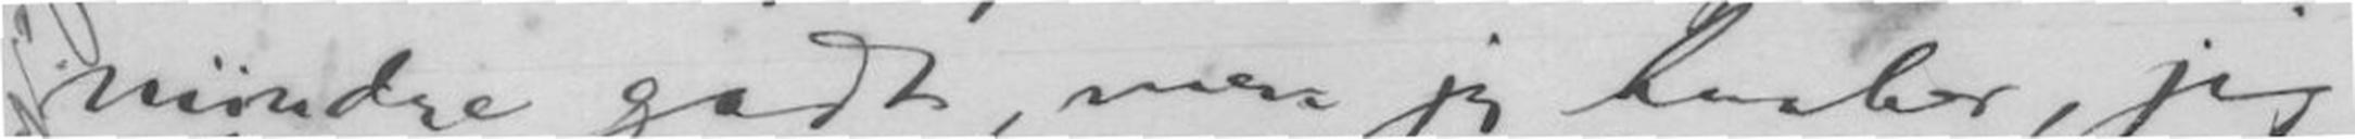mindre godt, men jeg haaber, jeg
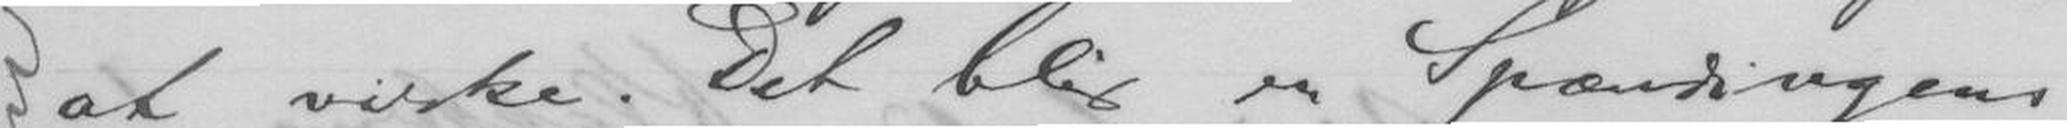at virke. Det blir en Spændingens
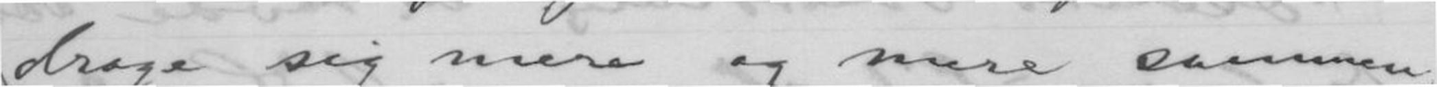drage sig mere og mere sammen,
